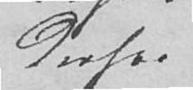Derfor
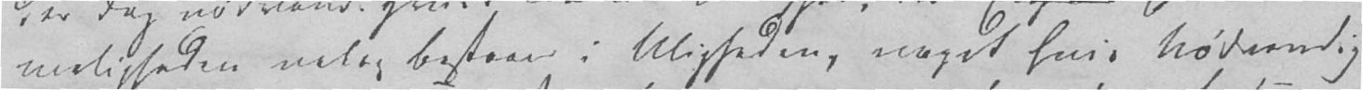meligheden netop bestaaer i Uligheden, noget hvis Nødvendig-
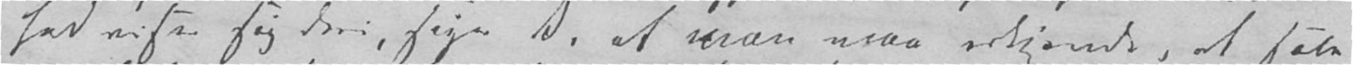hed viser sig deri, siger A. at man maa erkjende, at selv
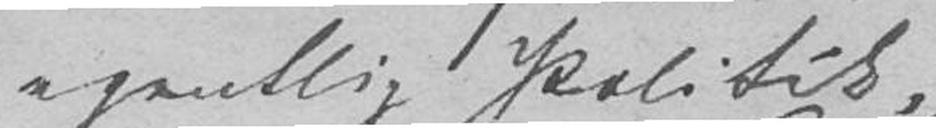egentlig Politik,
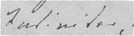Individer,
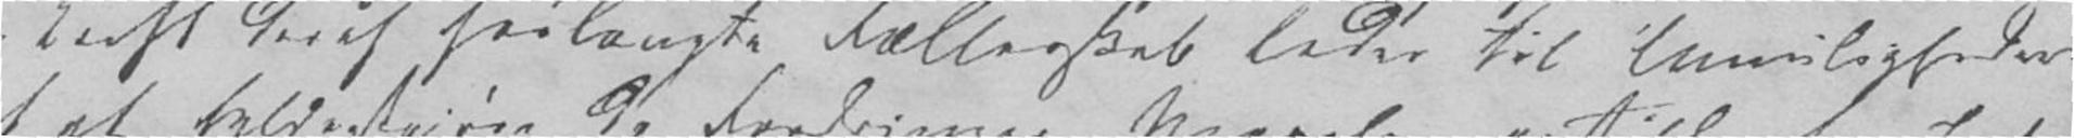i Kraft deraf forlangte Fællesskab leder til Umuligheden
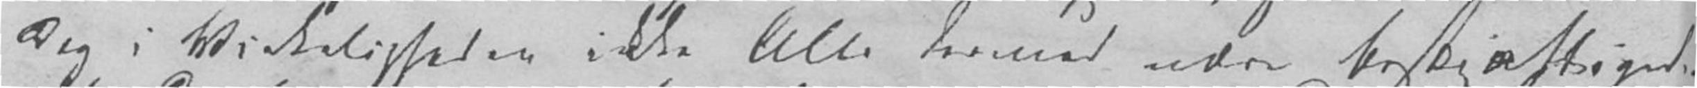dog i Virkeligheden ikke Alle dermed være beskjæftigede.
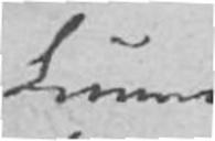kunne
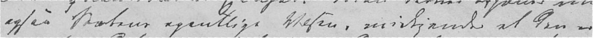ogsaa Statens egentlige Væsen, miskjender at den er
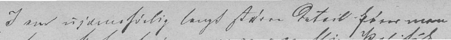I en ujævnførlig langt større Detail føres man
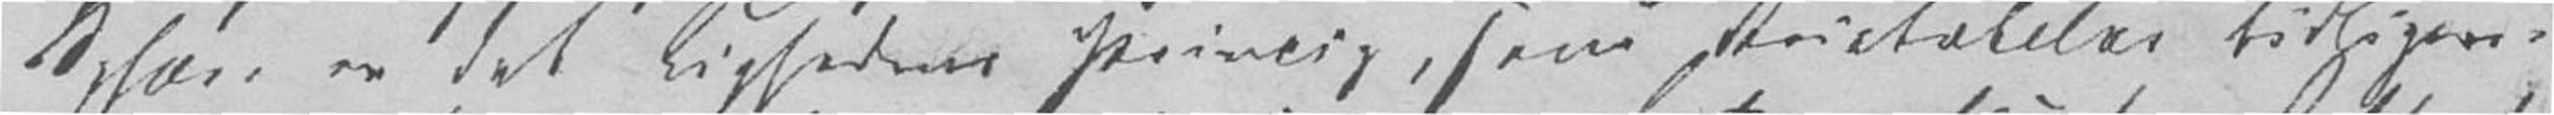Sphære er det Lighedens Princip, som Aristoteles tidligere i
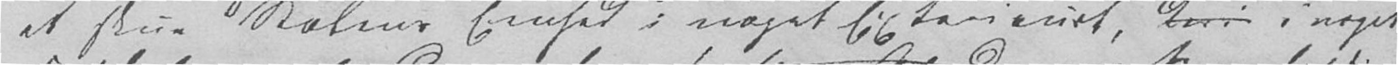at skue Statens Eenhed i noget Exterieurt, deri i noget
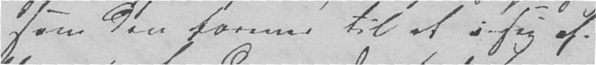som den forener til et i sig af-
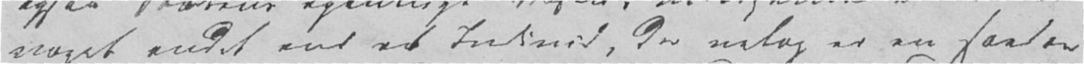noget andet end et Individ, der netop er en saadan
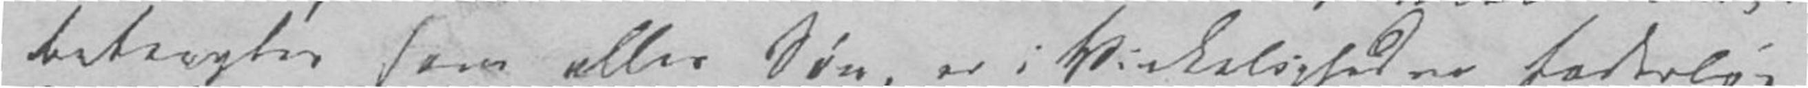betragtes som alles Søn, er i Virkelighed en faderløs,
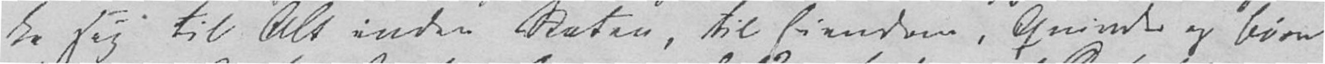ke sig til Alt inden Staten, til Eiendom, Qvinder og Børn
